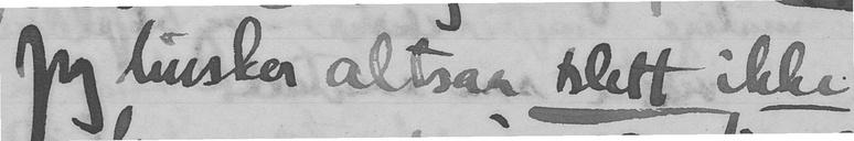Jeg husker altsaa slett ikke
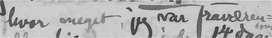hvor meget, jeg var fraværen-
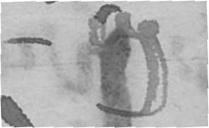- og
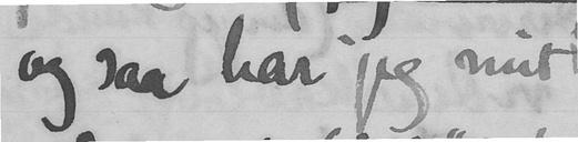og saa har jeg mit
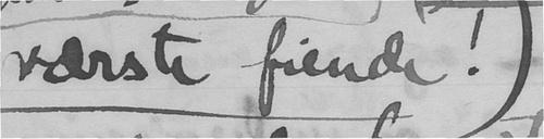værste fiende!)
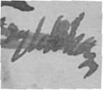sykdom
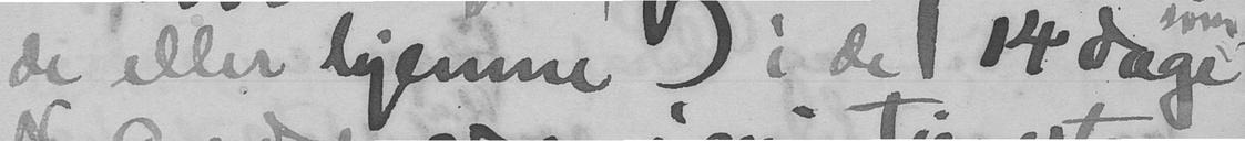de eller hjemme i de i 14 dage
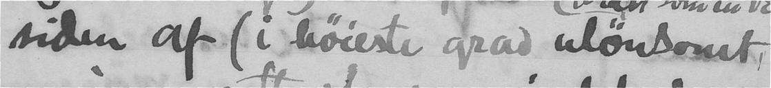siden af (i høieste grad ulønsomt,
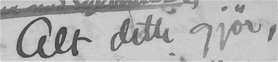Alt dette gjør,
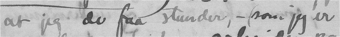at jeg de faa stunder, - (som jeg er
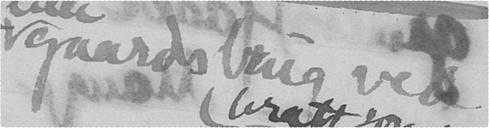gaardsbrug ved
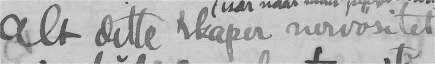Alt dette skaper nervøsitet
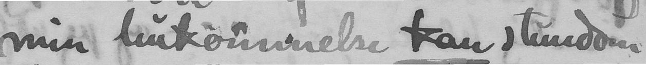min hukommelse kan stundom
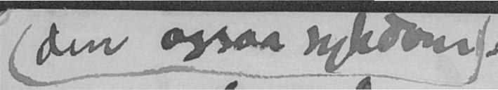(den ogsaa sykdom)
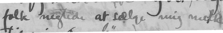folk negtede at sælge mig melk
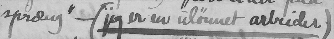spræng" - (jeg er en ulønnet arbeider)
